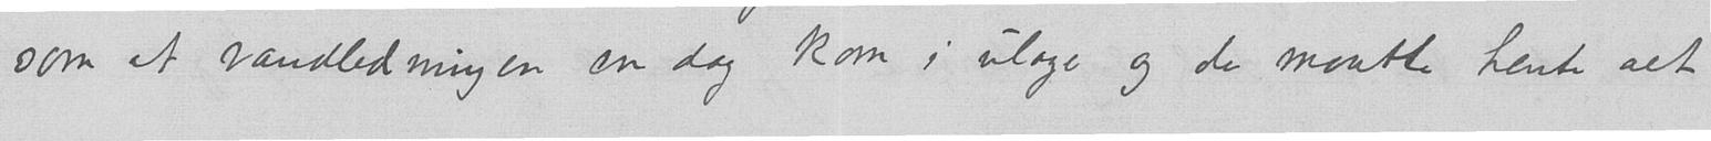som at vandledningen en dag kom i ulage og de maatte hente alt
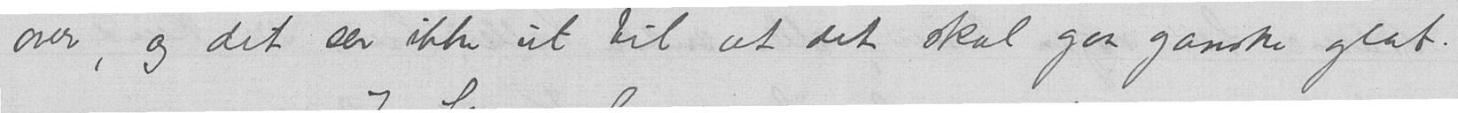over, og det ser ikke ut til at det skal gaa ganske glat.
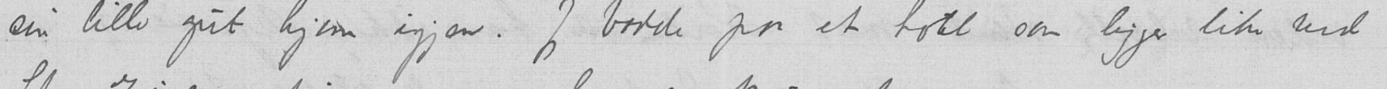sin lille gut hjem igjen. Jeg bodde paa et hotel som ligger like ved
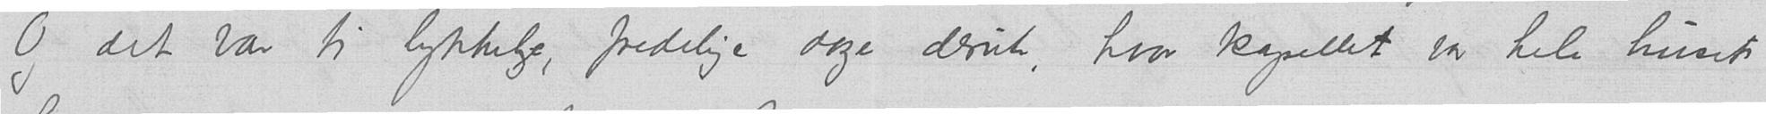Og det var ti lykkelige, fredelige dager derute, hvor kapellet var hele husets
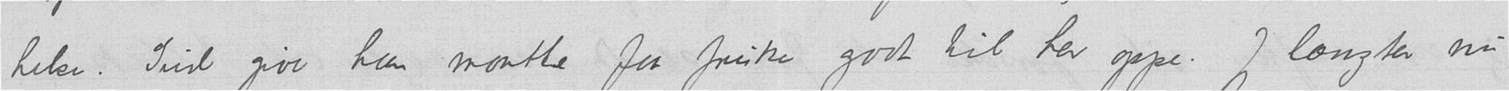helse. Gud give han maatte faa friske godt til her oppe. Jeg længter nu
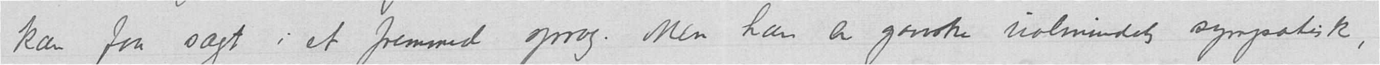kan faa sagt i et fremmed sprog. Men han er ganske ualmindelig sympatisk,
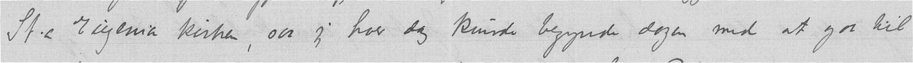St.a Eugenia kirken, saa jeg hver dag kunde begynde dagen med at gaa til
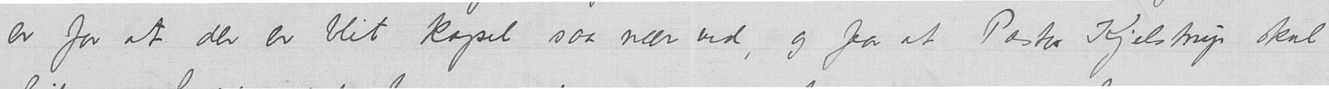er for at der er blit kapel saa nær ved, og for at Pastor Kjelstrup skal
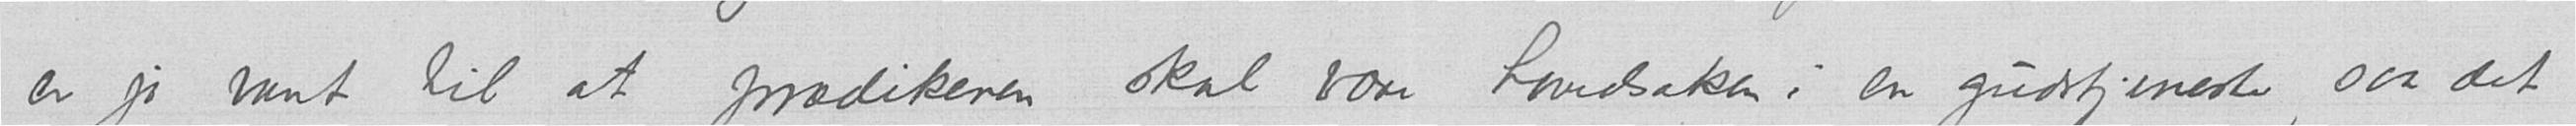er jo vant til at prædikenen skal være hovedsaken i en gudstjeneste, saa det
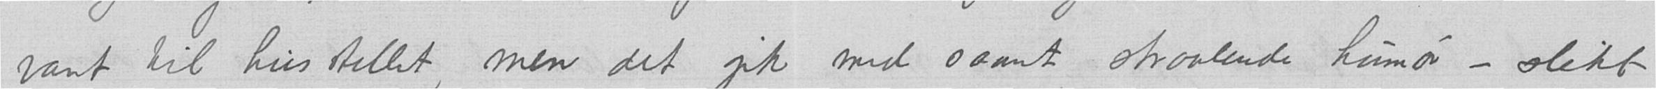vant til husstellet, men det gik med saant straalende humør - slikt
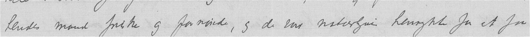hendes mand friske og fornøiede, og de var naturligvis henrykte for at faa
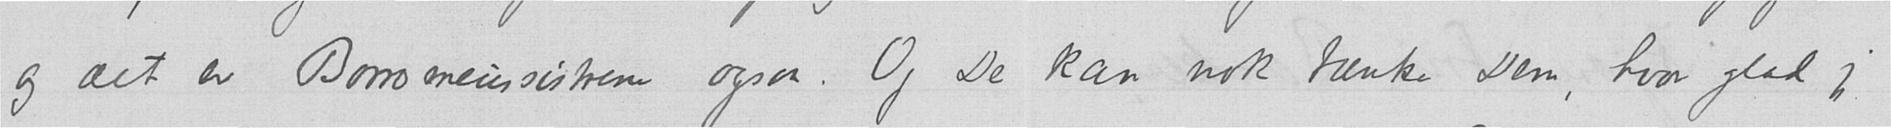og det er Borromeussøstrene ogsaa. Og De kan nok tænke Dem, hvor glad jeg
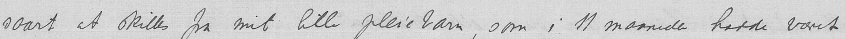saart at skilles fra mit lille pleiebarn, som i 11 maaneder hadde været
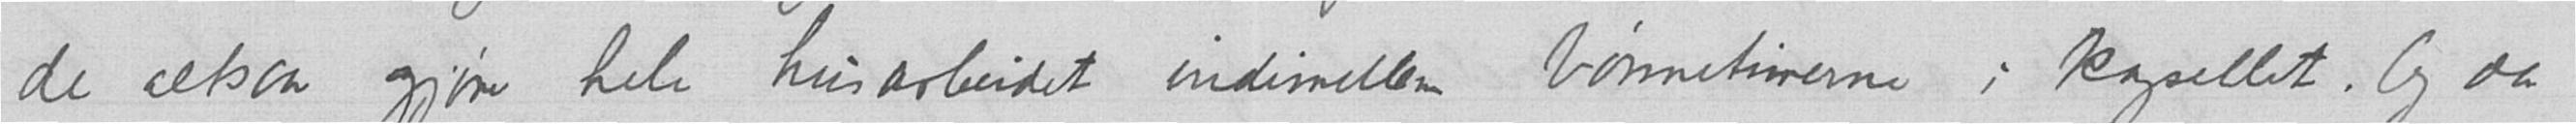de altsaa gjøre hele husarbeidet indimellem bønnetimerne i kapellet. Og da
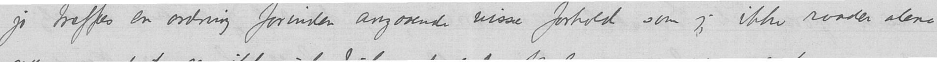jo træffes en ordning forinden angaaende visse forhold som jeg ikke raader alene
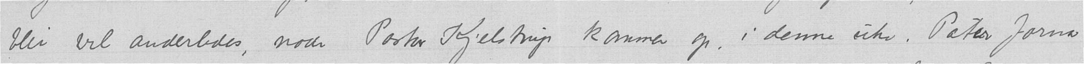blir vel anderledes, naar Pastor Kjelstrup kommer op, i denne uke. Pater Jorna
